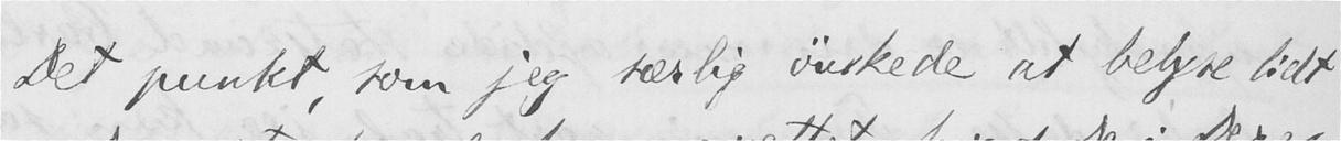Det punkt, som jeg særlig ønskede at belyse lidt
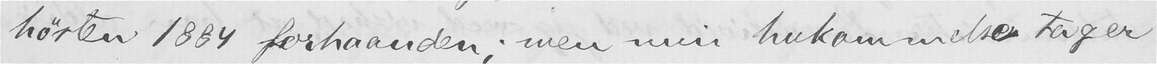høsten 1884 forhaanden; men min hukommelser tager
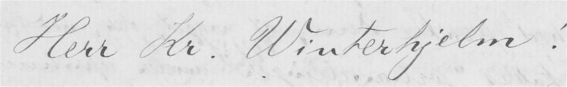Herr Kr. Winterhjelm!
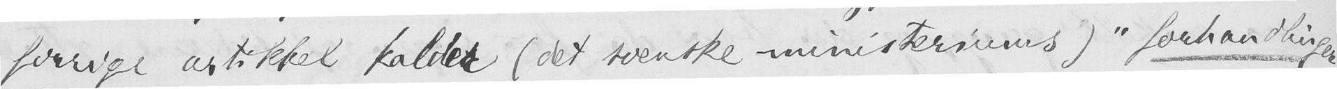forrige artikkel kalder (det svenske ministeriums) "forhandlinger
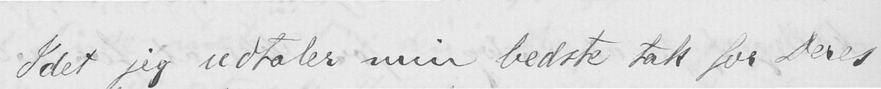Idet jeg udtaler min bedste tak for Deres
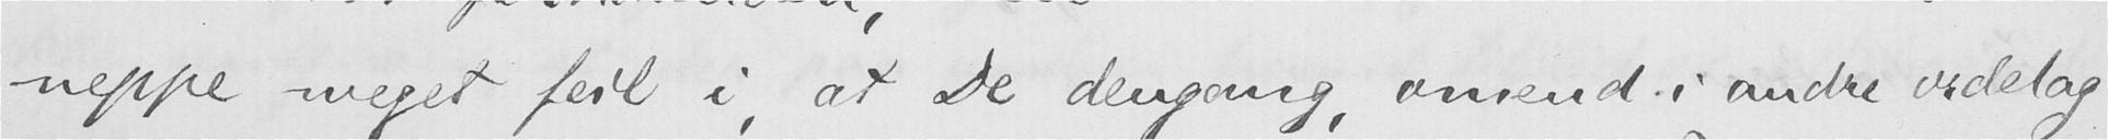neppe meget feil i, at De dengang, omend i andre ordelag
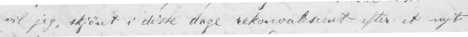vil jeg, skjønt i disse dage rekonvalescent efter et nyt
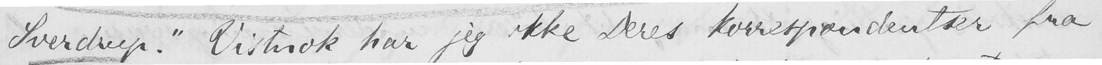Sverdrup." Vistnok har jeg ikke Deres korrespondentser fra
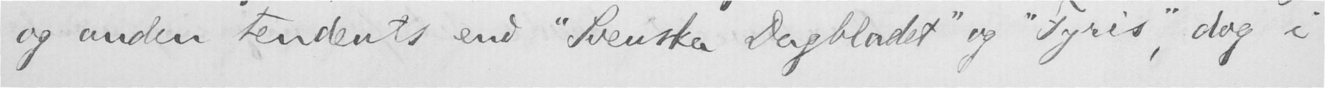og anden tendents end "Svenska Dagbladet" og "Fyris", dog i
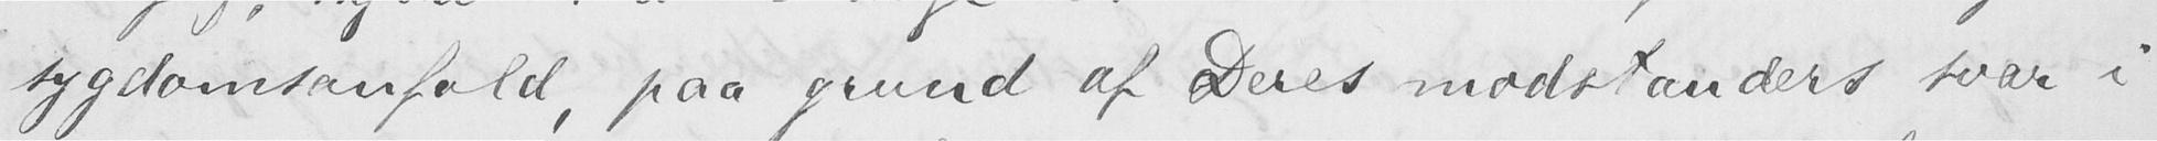sygdomsanfald, paa grund af Deres modstanders svar i
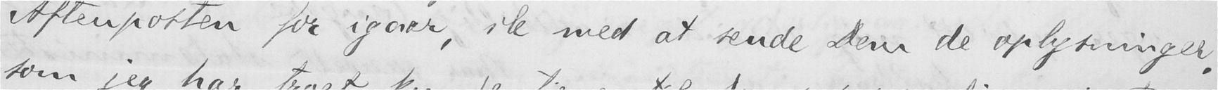Aftenposten for igaar, ile med at sende Dem de oplysninger,
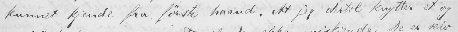kunnet kjende fra første haand. At jeg dertil knytter et og
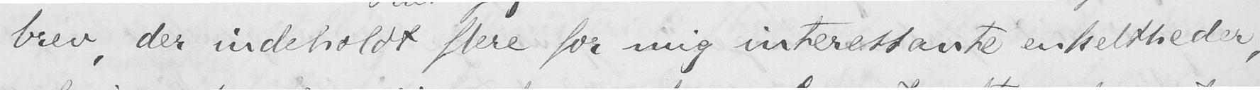brev, der indeholdt flere for mig interessante enkeltheder,
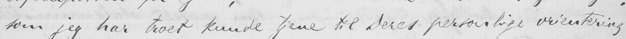som jeg har troet kunde tjene til Deres personlige orientering
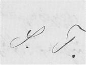S. T.
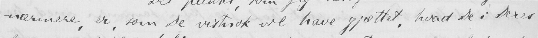nærmere, er, som De vistnok vil have gjættet, hvad De i Deres
